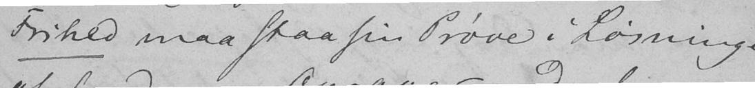Frihed maa staa sin Prøve i Løsningen
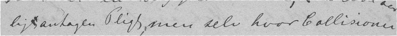ligt antagen Pligt, men selv hvor Collisionen
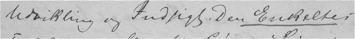Udvikling og Indsigt. Den Enkeltes
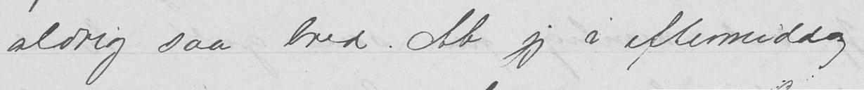aldrig saa bred. At jeg i eftermiddag
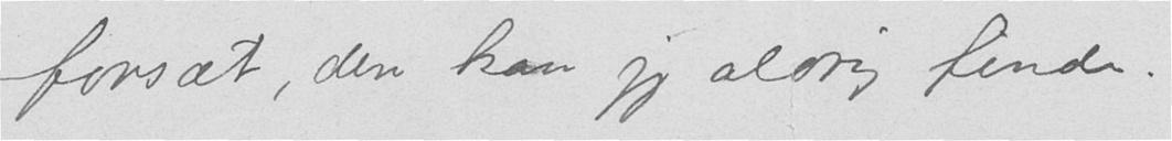forsæt, den kan jeg aldrig finde.
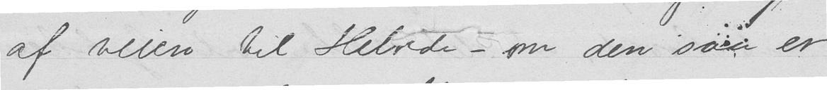af veien til Helvede - om den saa er
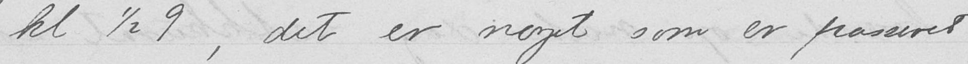kl 1/2 9, det er noget som er passeret
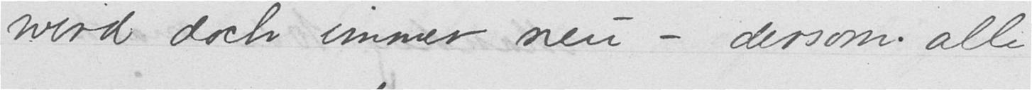wird doch immer neu - dersom alle
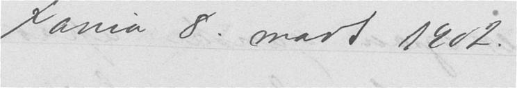Xania 8. marts 1902
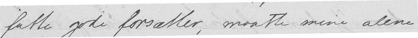fatte gode forsætter, maatte mine alene
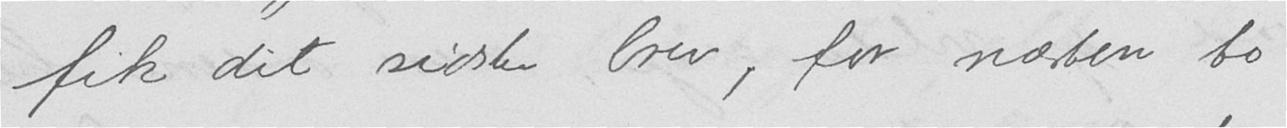fik dit sidste brev, for næsten to
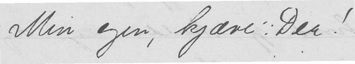Min egen, kjære Dea!
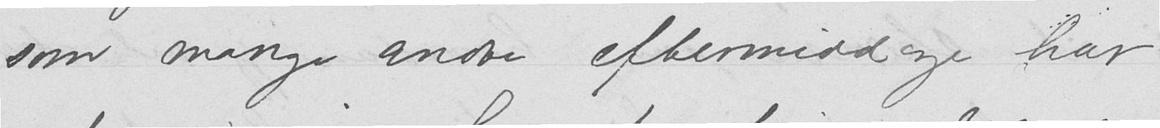som mange andre eftermiddage har
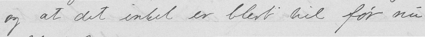og at det intet er blevet til før nu
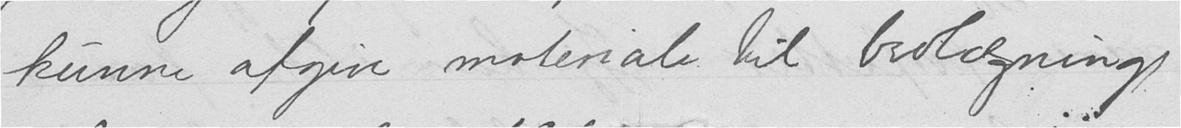kunne afgive materiale til brolægning
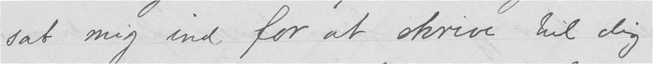sat mig ind for at skrive til dig
